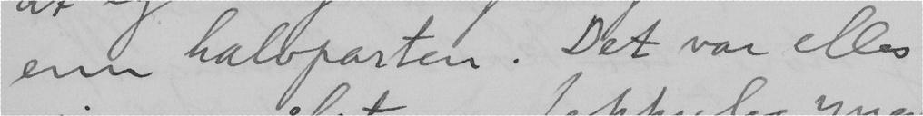enn halvparten. Det var elles
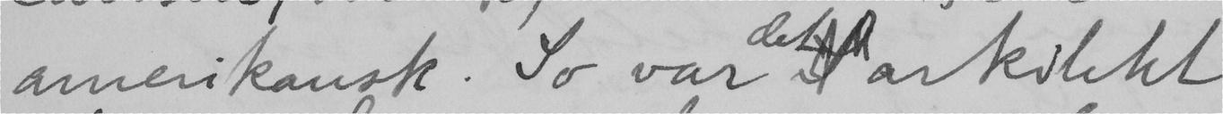amerikansk. So var det arkitekt
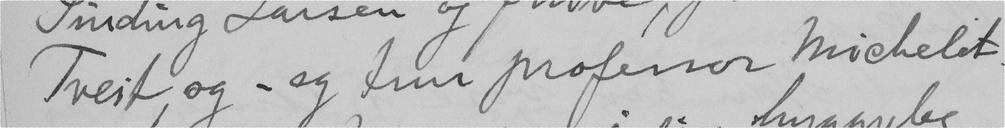Tveit, og – eg trur professor Michelet.
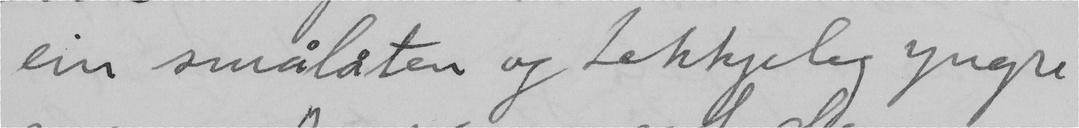ein smålåten og tekkjeleg yngre
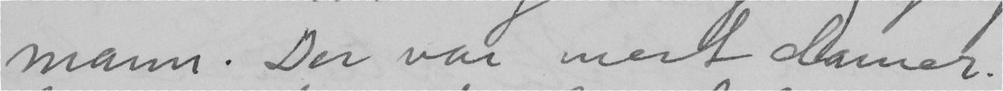mann. Der var mest damer.
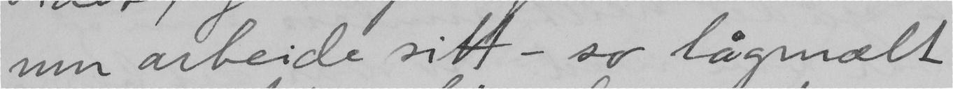um arbeide sitt – so lågmælt
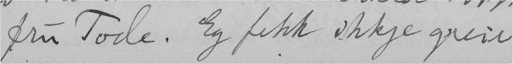fru Tode. Eg fekk ikkje greie
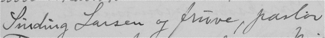Sinding Larsen og fruve, pastor
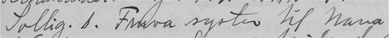Sollig. I. Fruva syster til Nana
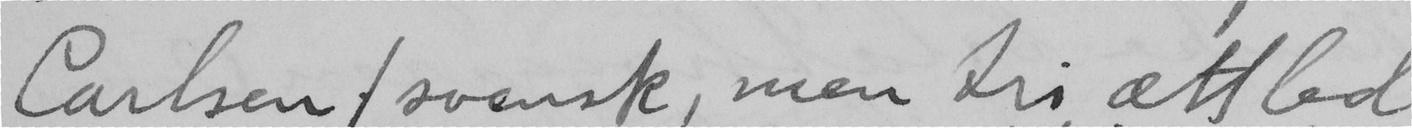Carlsen / svensk, men tri ættled
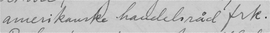amerikanske handelsråd frk.
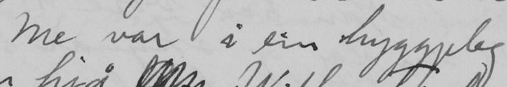Me var i ein hyggjeleg
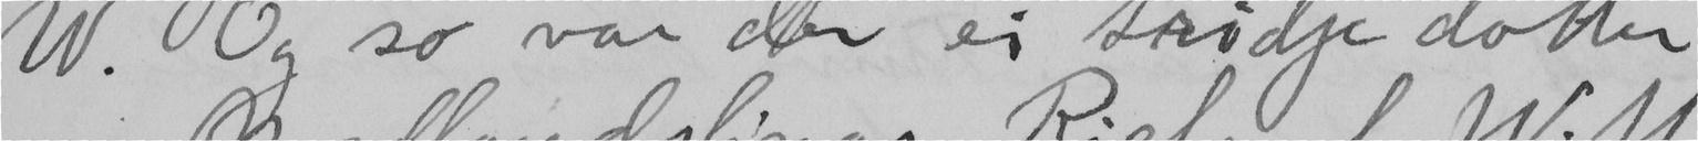W. Og so var der ei tridje dotter
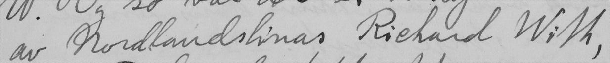av Nordlandslinas Richard With,
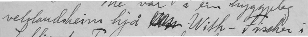velstandsheim hjå  With-Fischer i
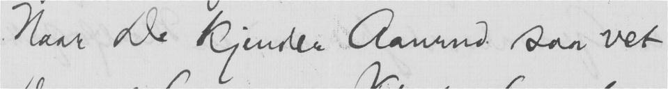Naar De kjender Aanrud saa vet
